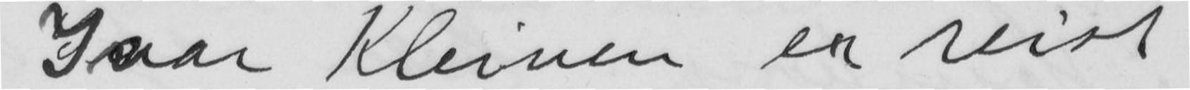Ivar Kleiven er reist
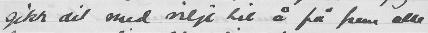gikk dit med vilje til å få frem alle
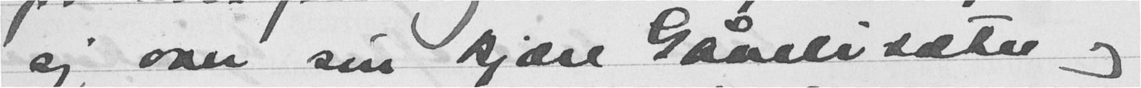sig over sin kjære Gåneli sæter og
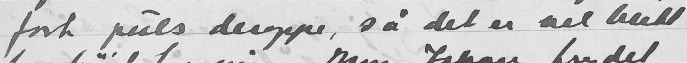fort puls deroppe, så det er vel blitt
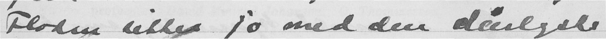Floden sitter jo med den dårligste
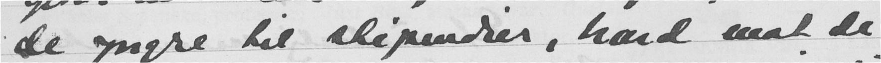de yngre til stipendier, hard mot de
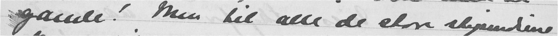gamle! Men til alle de store stipendiene
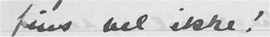fins vel ikke!
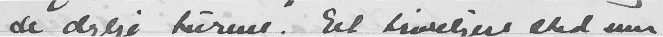de deilige turene. Et triveligere sted enn
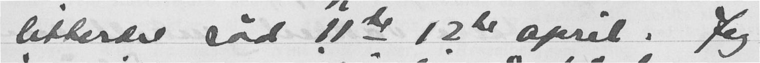litterære råd 11de 12de april. Jeg
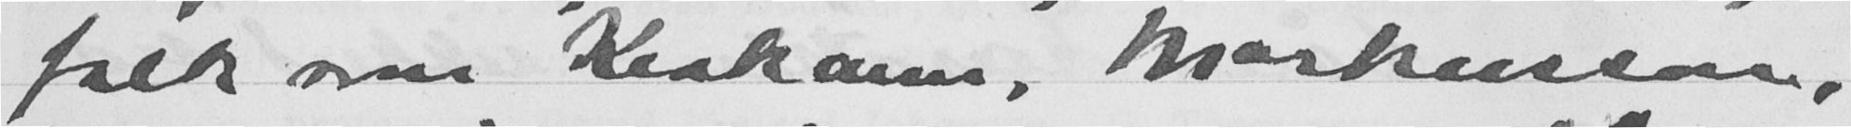folk som Krokann, Markusson,
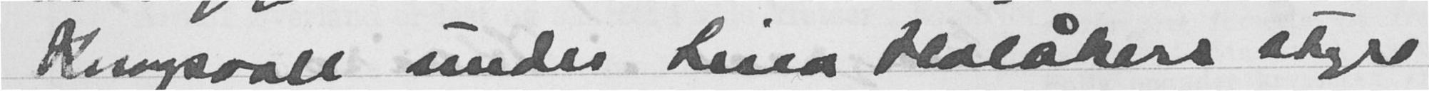Kongsvoll under Lina Holåkers styre
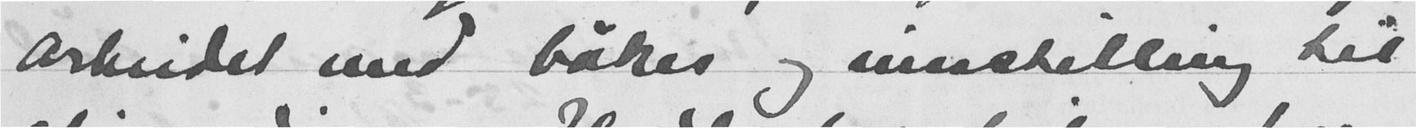arbeidet med bøker og innstilling til
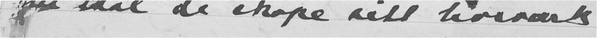nu skal de skape sitt korverk
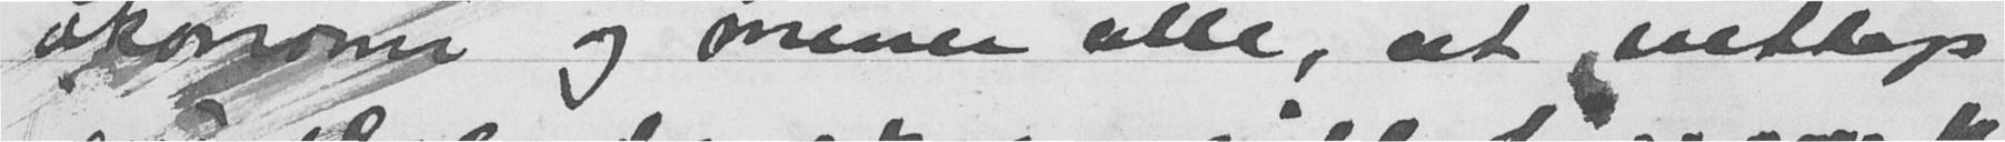økonomi og mener alle, at nettop
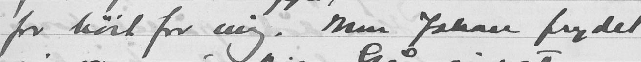for høit for mig. Men Johan frydet
